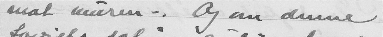mot muren -. Og om denne
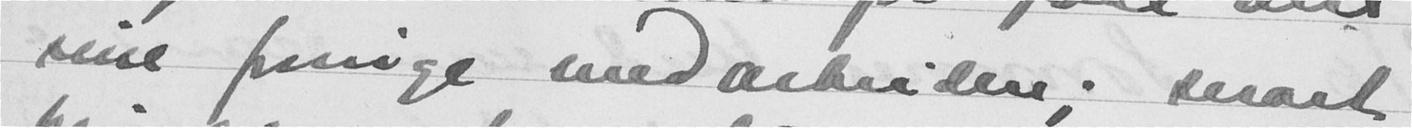sine forrige medarbeidere; snart
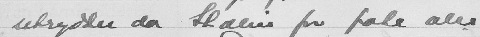utrydder da Stalin fra fote alle
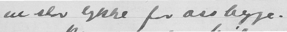en stor lykke for oss begge.
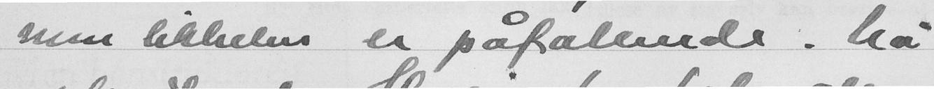men likheten er påfallende. Nå
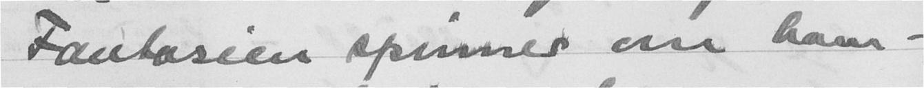Fantasien spinner om ham -
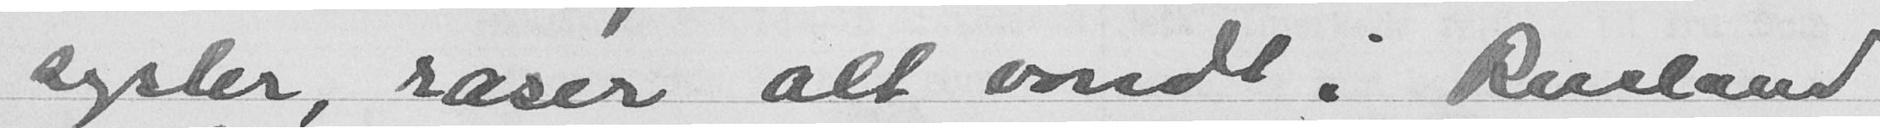sysler, raser alt vondt i Rusland
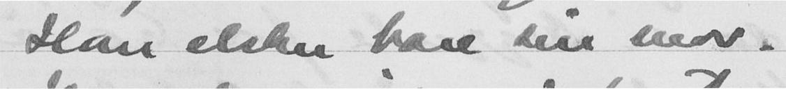Han elsker bare sin mor.
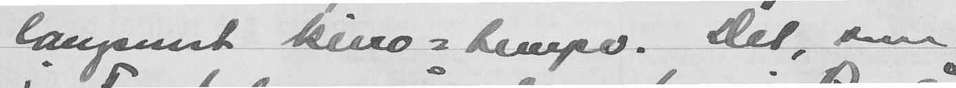langsomt kino-tempo. Det, som
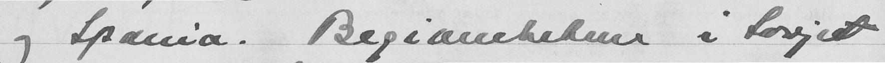og Spania. Begivenhetene i Sovjet
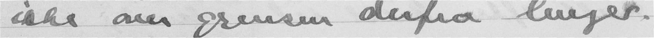ikke over grensen derfra lenger.
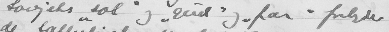Sovjets "sol" og "gud" og "far" forlyder
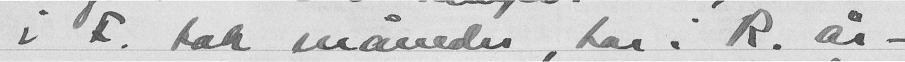i F. tok måneder, tar i R. år -
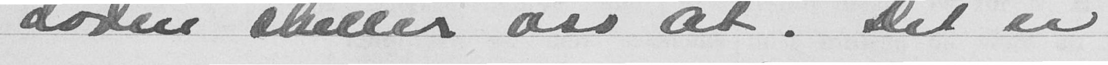døden skiller oss at. Det er
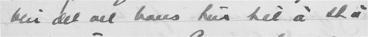blir det vel hans tur til å stå
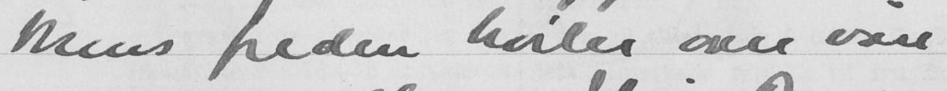Mens freden hviler over våre
